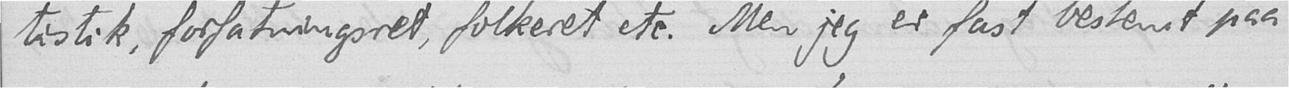tistik, forfatningsret, folkeret etc. Men jeg er fast bestemt paa
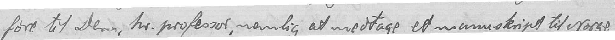føre til Dem, hr. professor, nemlig at medtage et manuskript til Norge,
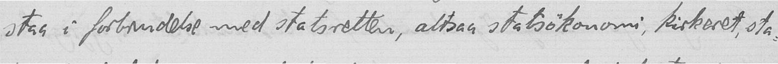staa i forbindelse med statsretten, altsaa statsøkonomi, kirkeret, sta-
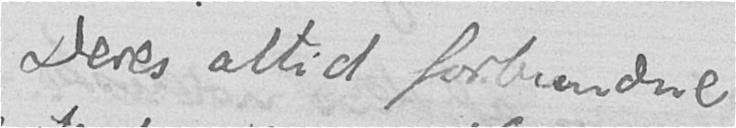Deres altid forbundne
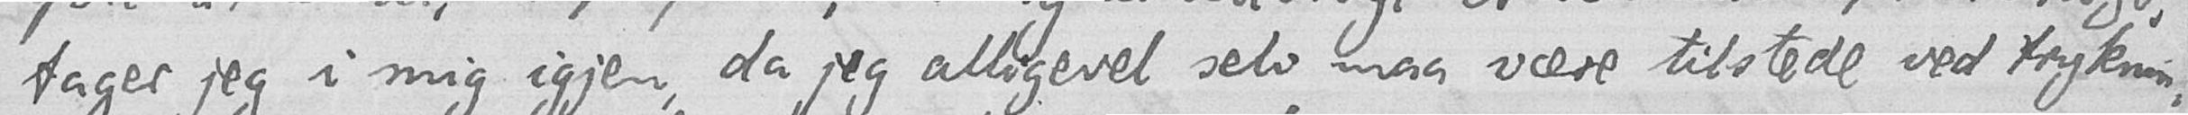tager jeg i mig igjen, da jeg alligevel selv maa være tilstede ved tryknin-
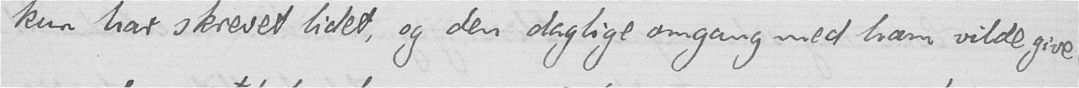kun har skrevet lidet, og den daglige omgang med ham vilde give
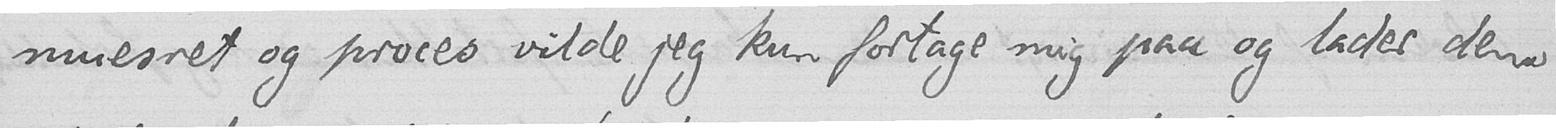muesret og proces vilde jeg kun fortage mig paa og lader dem
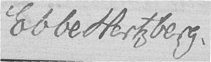Ebbe Hertzberg.
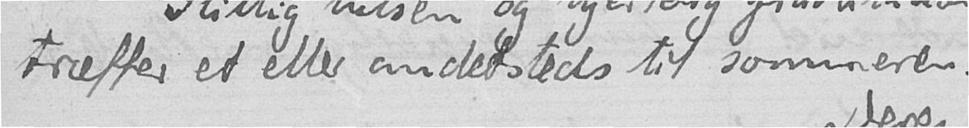træffer et eller andetsteds til sommeren.
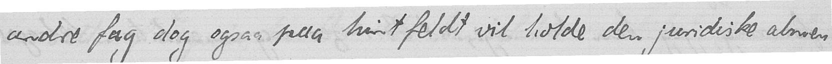andre fag dog ogsaa paa hint feldt vil holde den juridiske almen-
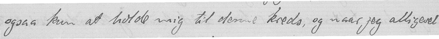ogsaa kun at holde mig til denne kreds, og naar jeg alligevel
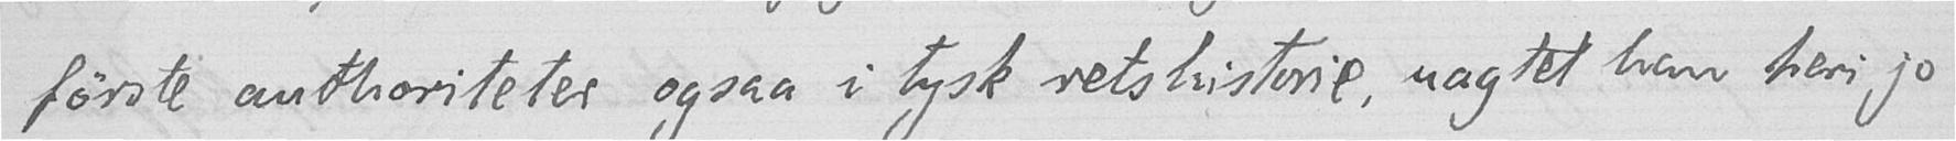første authoriteter ogsaa i tysk retshistorie, uagtet han heri jo
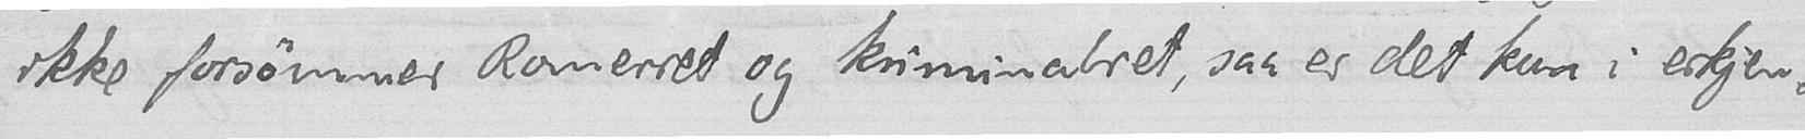ikke forsømmer Romerret og kriminalret, saa er det kun i erkjen-
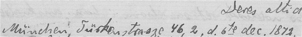München, Türkenstrasse 46, 2, d. 6te dec. 1872.
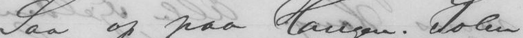Saa op paa Haugen. Solen
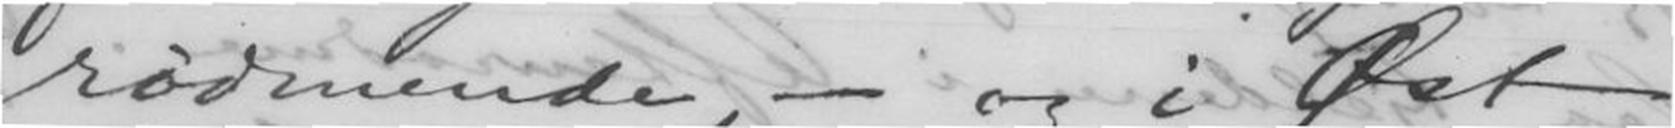rødmende, - og i Øst
